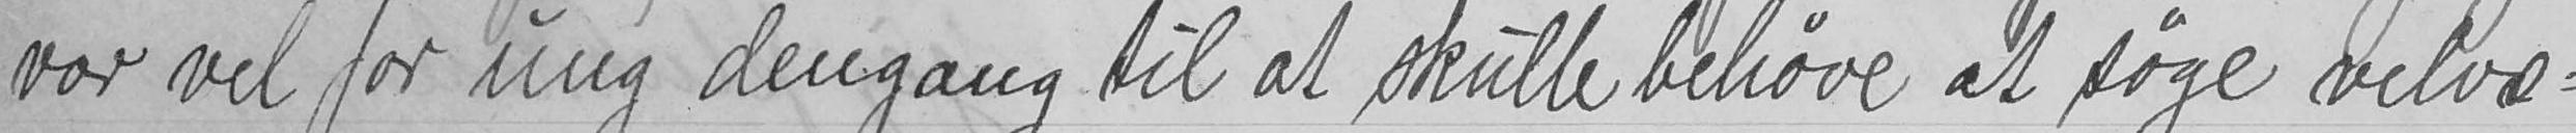var vel for ung dengang til at skulle behøve at søge velvæ-
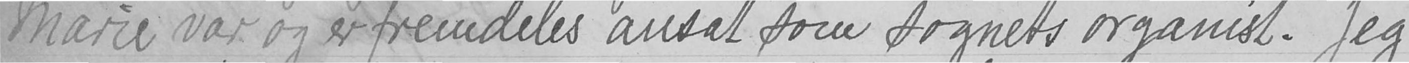Marie var og er fremdeles ansat som sognets organist. Jeg
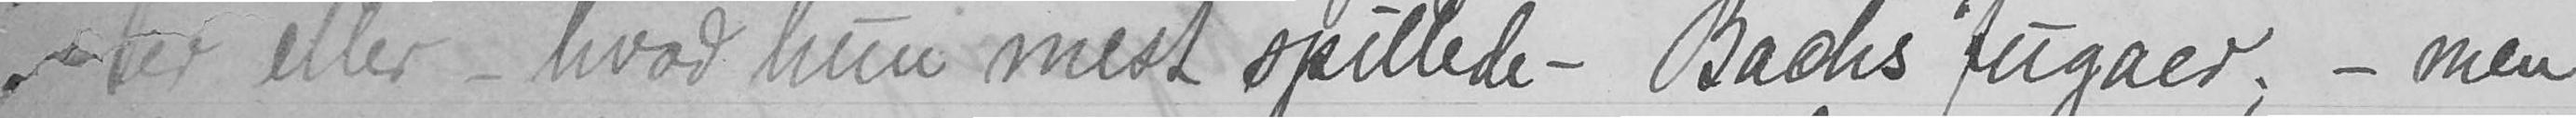ter eller - hvad hun mest spillede - Bachs fugaer; - men
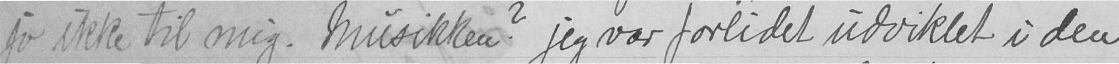jo ikke til mig. Musikken? jeg var forlidet udviklet i den
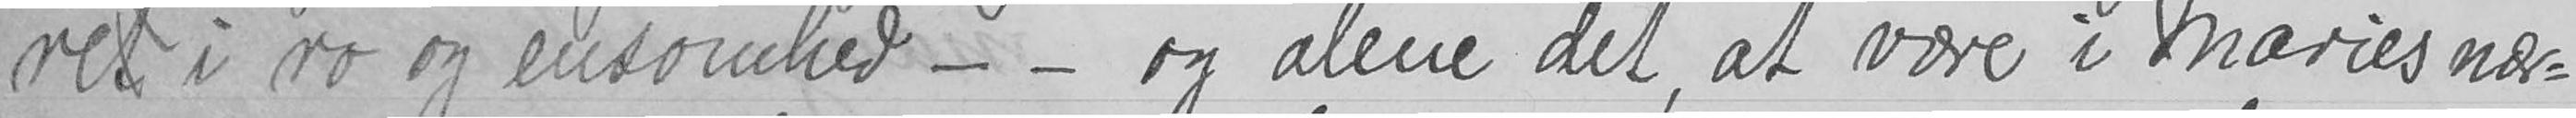ret i ro og ensomhed - - og alene det, at være i Maries nær-
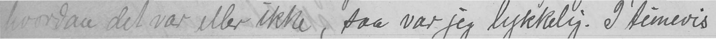hvordan det var eller ikke, saa var jeg lykkelig. I timevis
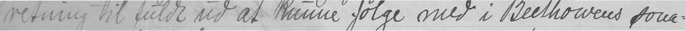retning til fuldt ud at kunne følge med i Beethovens sona-
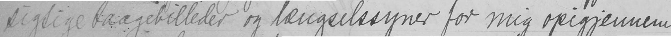sigtige taagebilleder og længselssyner for mig opigjennem
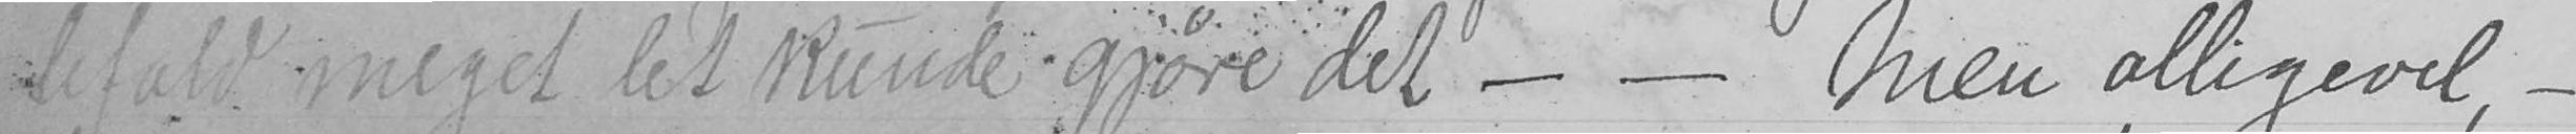lefald meget let kunde  gjøre det - - Men alligevel, -
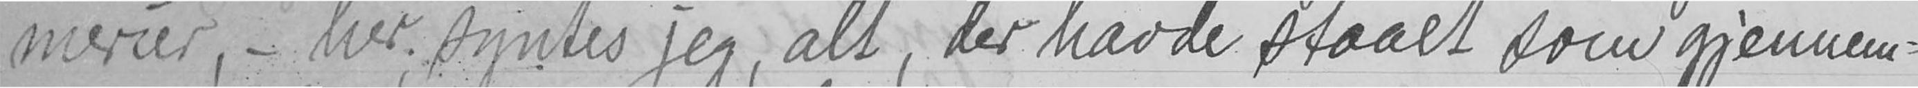merier, her syntes jeg, alt, der havde staaet som gjennem-
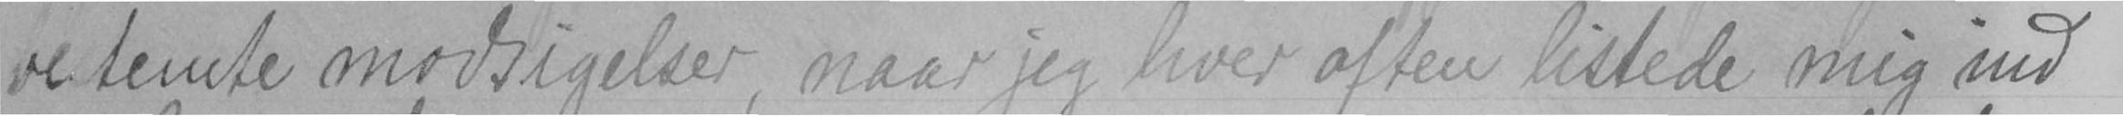bestemte modsigelser, naar jeg hver aften listede mig ind
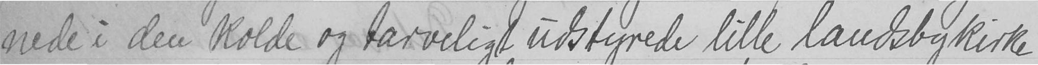nede i den kolde og tarveligt udstyrede lille landsbykirke
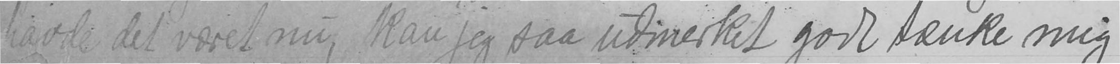havde det været nu, kan jeg saa udmerket godt tænke mig
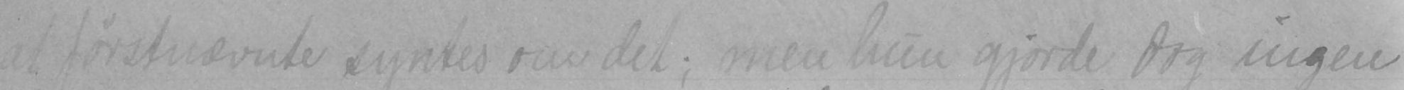at førstvævnte syntes om det; men hun gjorde ingen
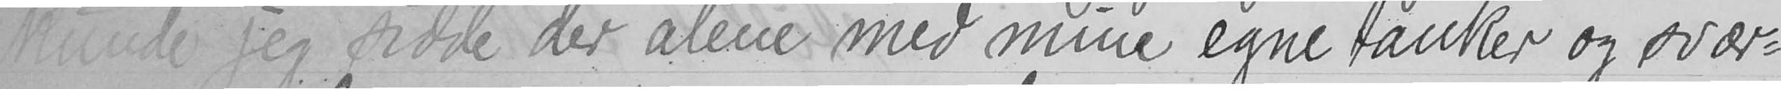kunde jeg sidde der alene med mine egne tanker og svær-
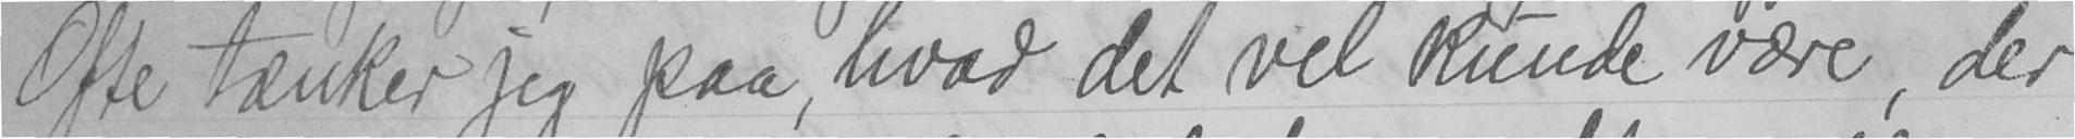Ofte tænker jeg paa, hvad det vil kunde være, der
